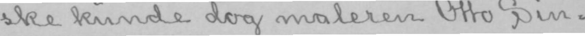ske kunde dog maleren Otto Sin-
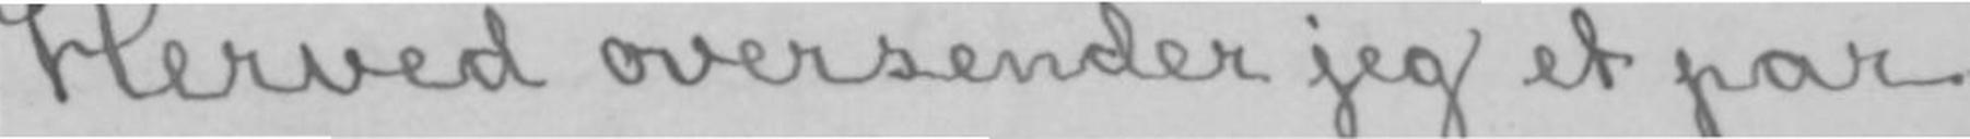Herved oversender jeg et par
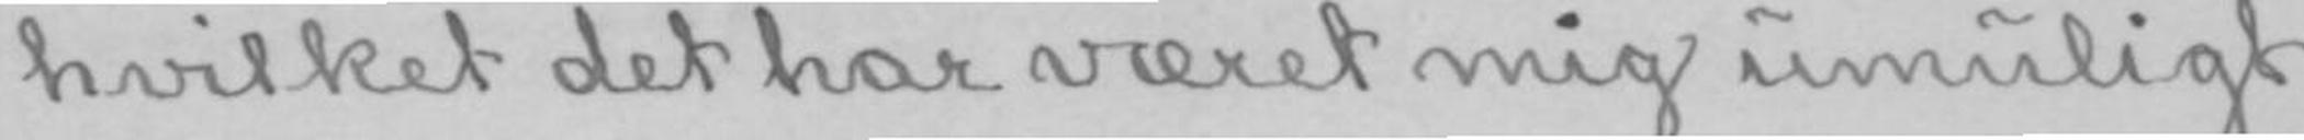hvilket det har været mig umuligt
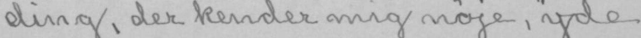ding, der kender mig nøje, yde
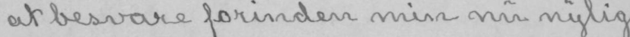at besvare forinden min nu nylig
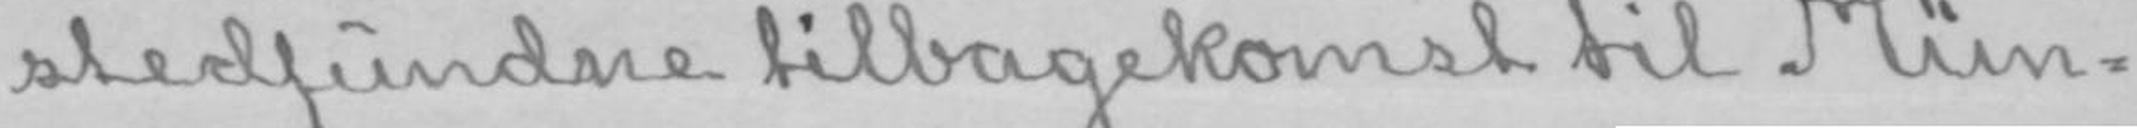stedfundne tilbagekomst til Mün-
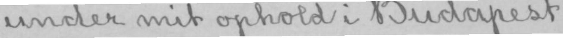under mit ophold i Budapest
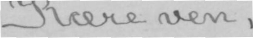Kære ven,
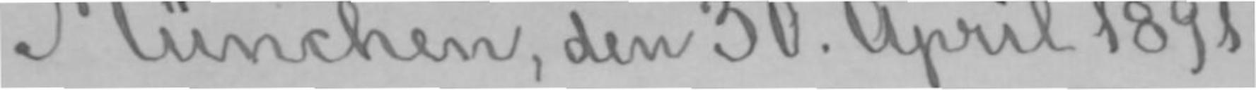München, den 30. April 1891.
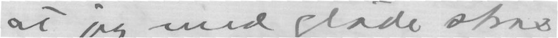at jeg med glæde strax
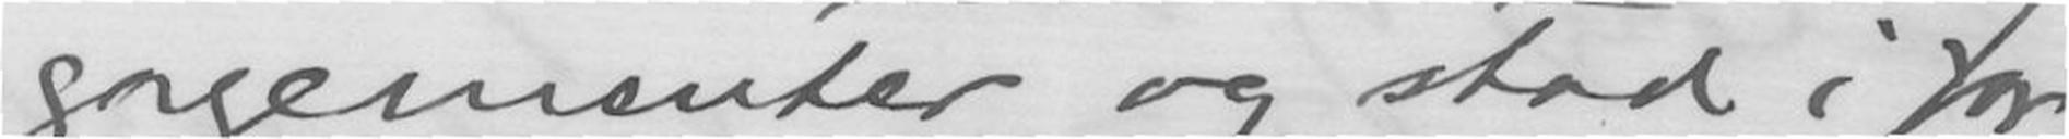gagementer og stod i for-
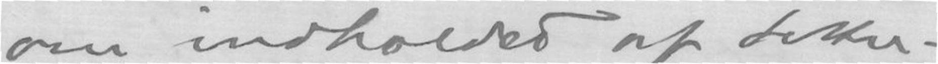om indholdet af sekre-
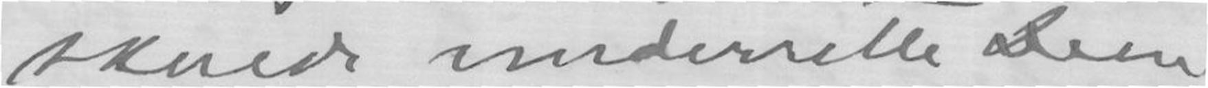skulde underrette Dem
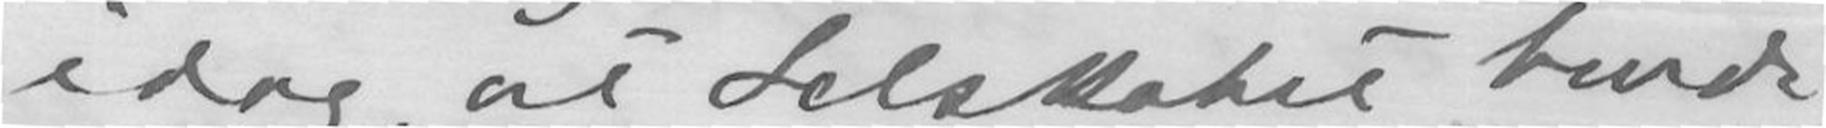idag, at Selskabet burde
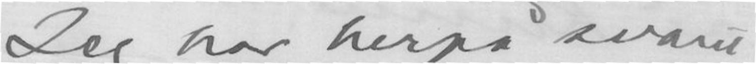Jeg har herpå svaret
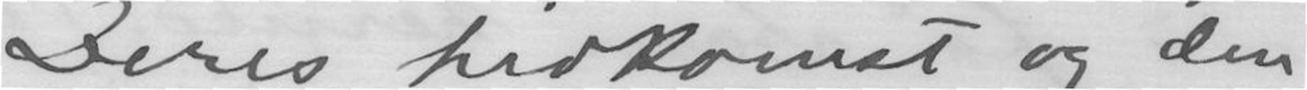Deres hidkomst og den
